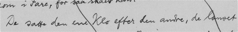De satte den ene Klo efter den andre, de lænset
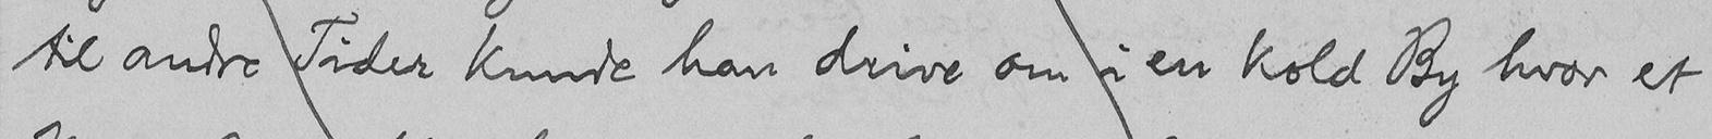til andre Tider kunde han drive om i en kold By hvor et
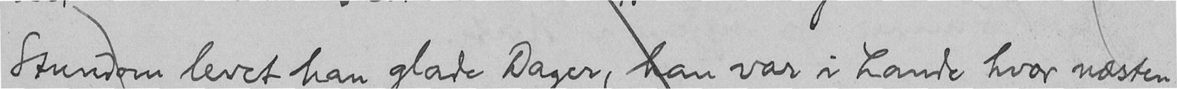Stundom levet han glade Dager, han var i Lande hvor næsten
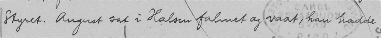Styret. August sat i Halsen falmet og vaat, han hadde
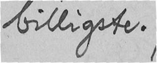billigste.
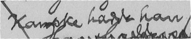Kanske hadde han
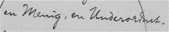en Menig, en Underordnet.
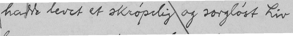hadde levet et skrøpelig og sorgløst Liv
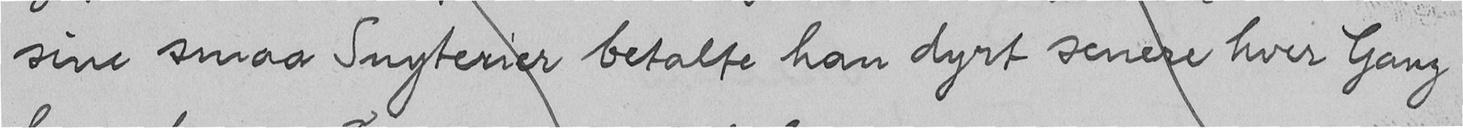sine smaa Snyterier betalte han dyrt senere hver Gang
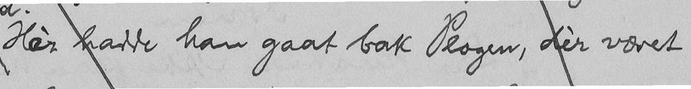Hèr hadde han gaat bak Plogen, dèr været
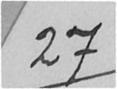27
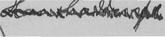Handerldasel
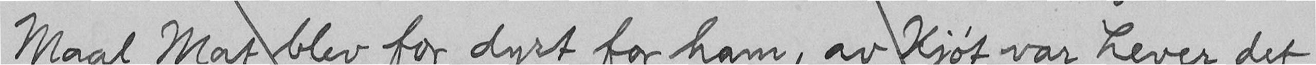Maal Mat blev for dyrt for ham, av Kjøt var Lever det
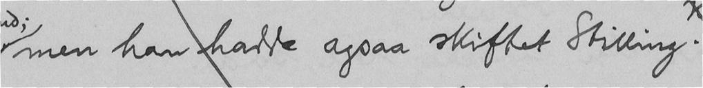men han hadde ogsaa skiftet Stilling.
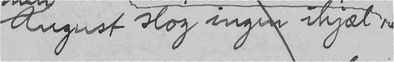August slog ingen ihjæl,
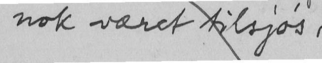nok været tilsjøs,
